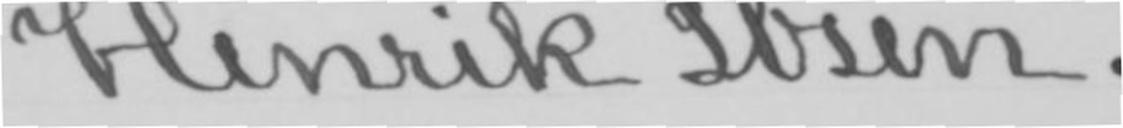Henrik Ibsen.
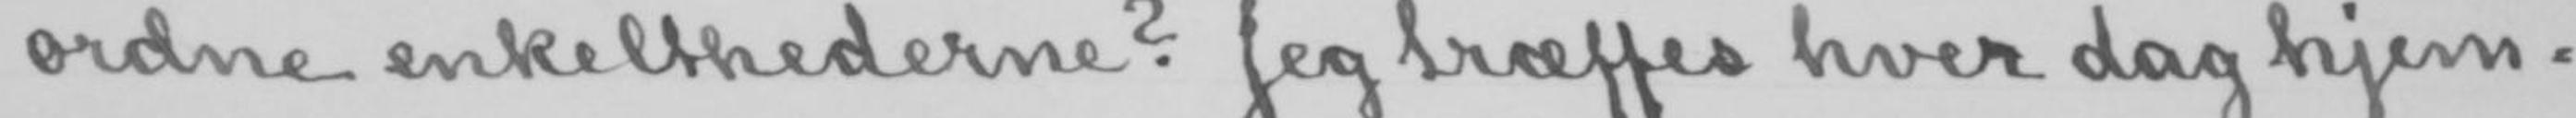ordne enkelthederne? Jeg træffes hver dag hjem-
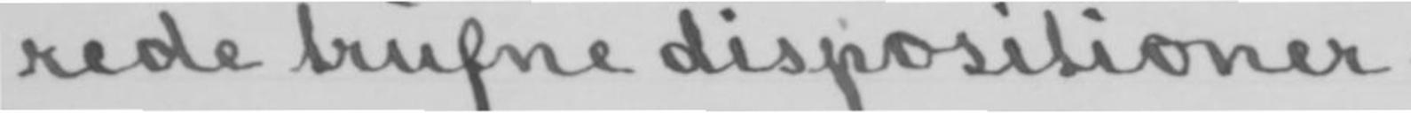rede trufne dispositioner.
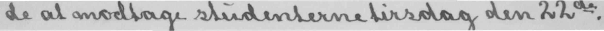de at modtage studenterne tirsdag den 22de.
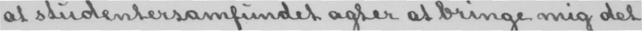at studentersamfundet agter at bringe mig det
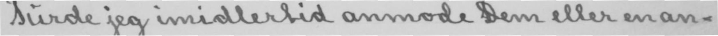Turde jeg imidlertid anmode Dem eller en an-
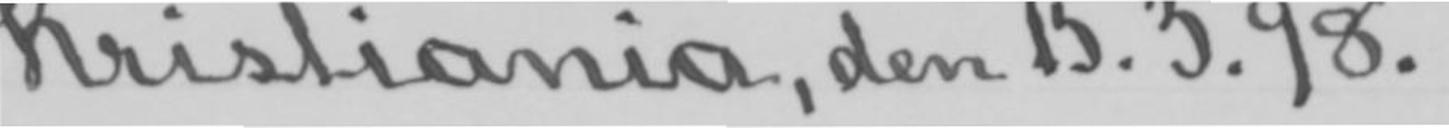Kristiania, den 15.3.98.
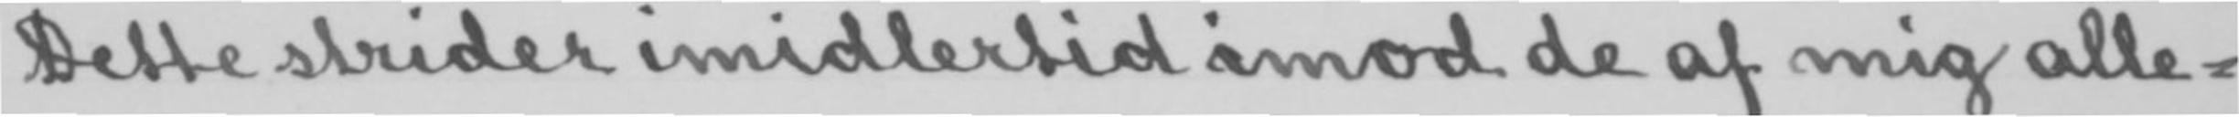Dette strider imidlertid imod de af mig alle-
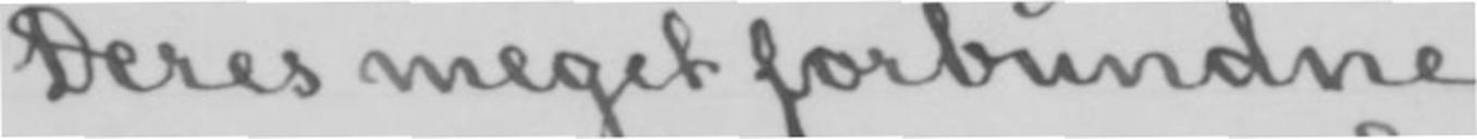Deres meget forbundne
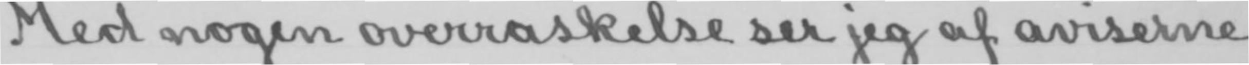Med nogen overraskelse ser jeg af aviserne
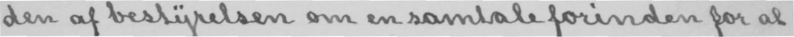den af bestyrelsen om en samtale forinden for at
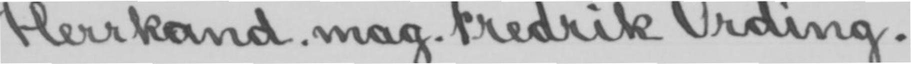Herr kand. mag. Fredrik Ording.
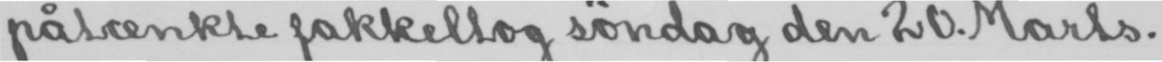påtænkte fakkeltog søndag den 20. Marts.
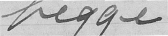begge
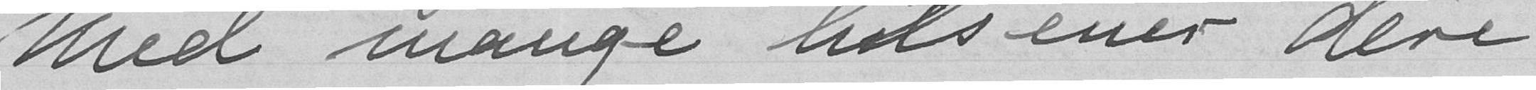Med mange hilsener dere
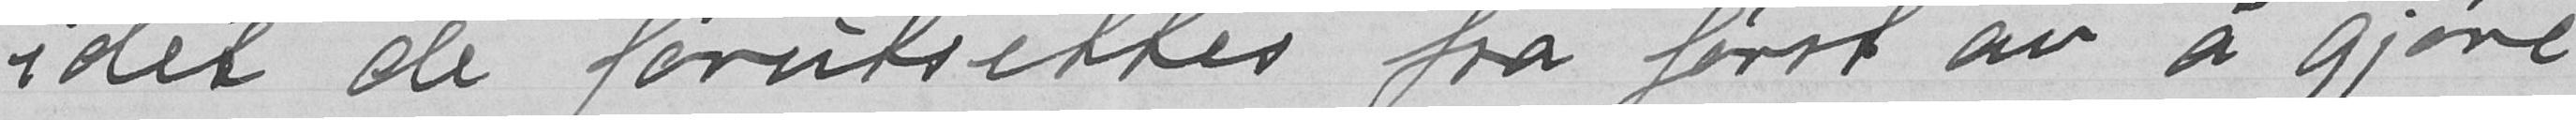idet de forutsettes fra først av å gjøre
